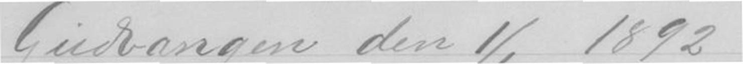Gudvangen den 1/1 1892
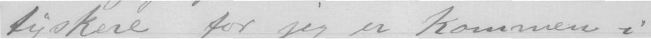tyskere for jeg er kommen i
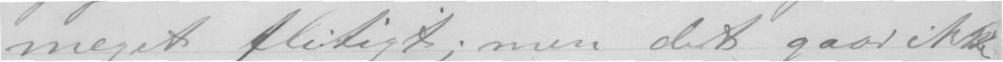meget flitigt; men det gaar ikke
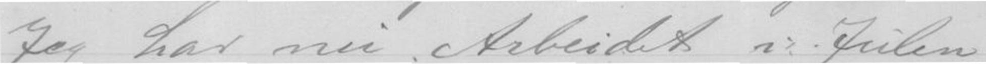Jeg har nu Arbeidet i Julen
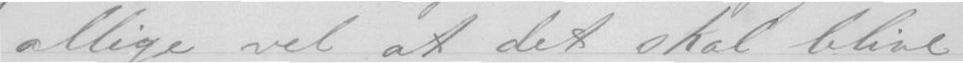allige vel at det skal blive
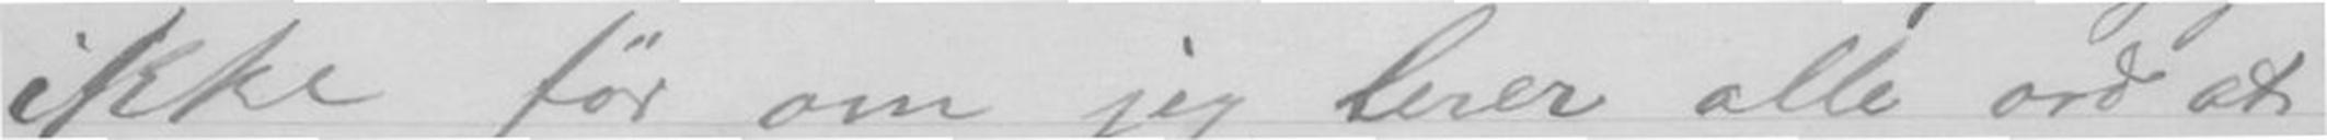ikke før om jeg lerer alle ord at
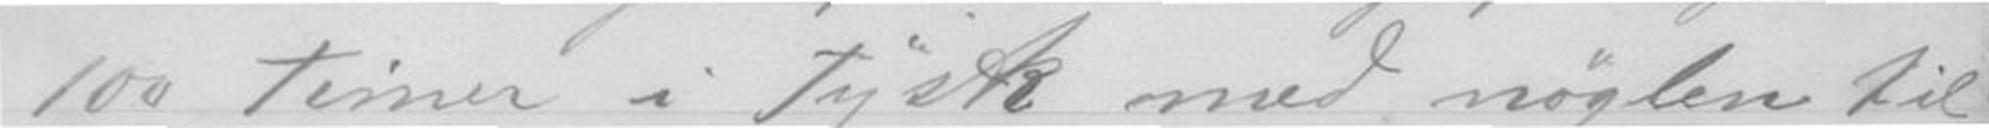100 timer i Tysk med noglen til
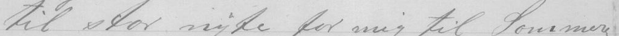til stor nyte for mig til Sommer,
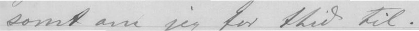somt om jeg for thid til.
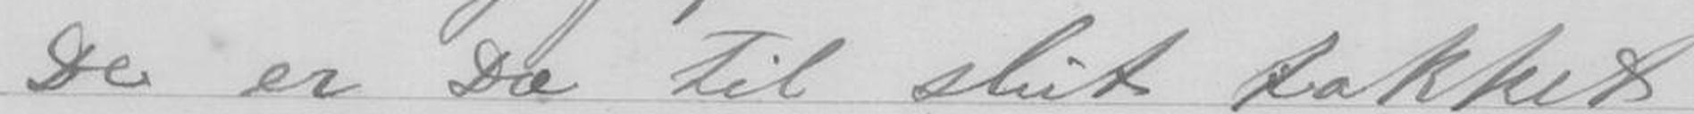De er Da til slut takket
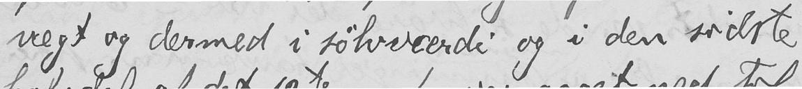vægt og dermed i sølvværdi og i den sidste
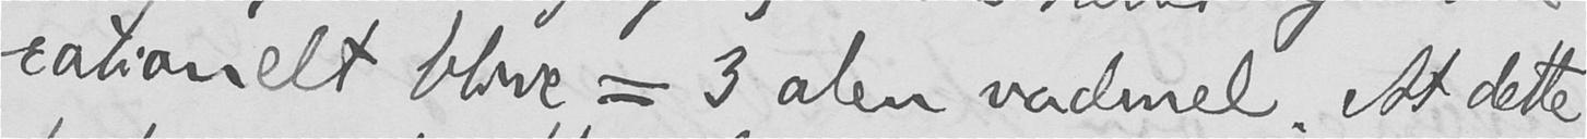rationelt blive = 3 alen vadmel. At dette
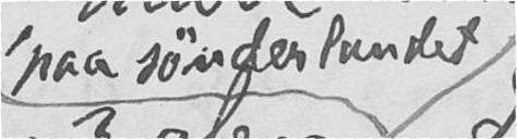paa sønderlandet
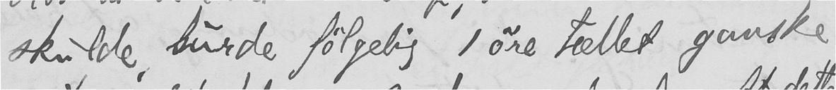skulde, burde følgelig 1 øre tællet ganske
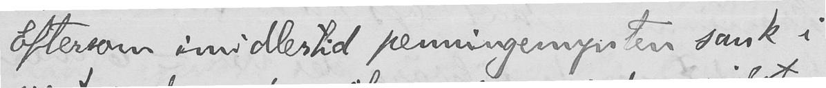Eftersom imidlertid penningemynten sank i
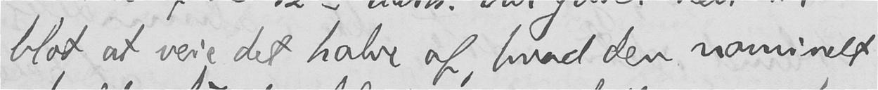blot at veie det halve af, hvad den nominelt
